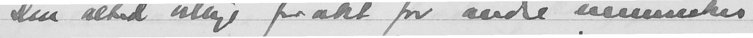Den alltid villige forakt for andre mennesker
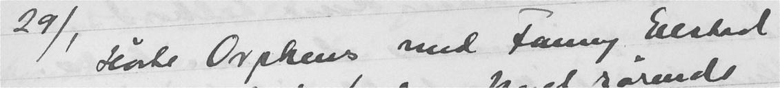29/1 Hørte Orpheus med Fanny Elstad
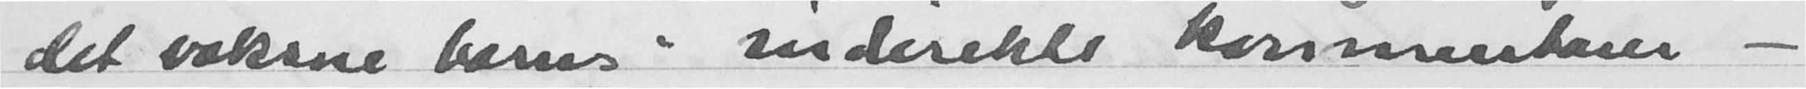det voksne barns indirekte kommentarer -
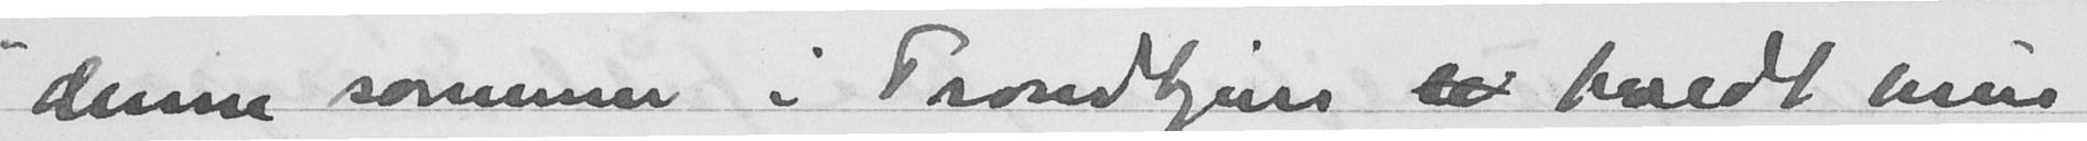denne sommeren i Trondhjem  holdt hun
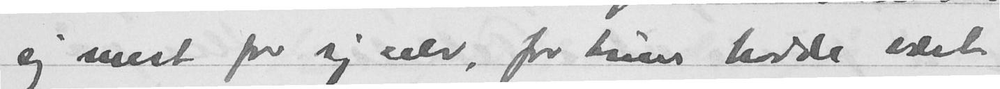sig mest for sig selv, for hun hadde vært
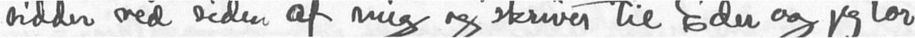sidder ved siden af mig og skriver til Eder og jeg tør
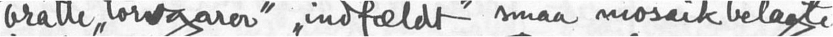bratte "torvgarer" "indfældt" smaa mosaikbelagte
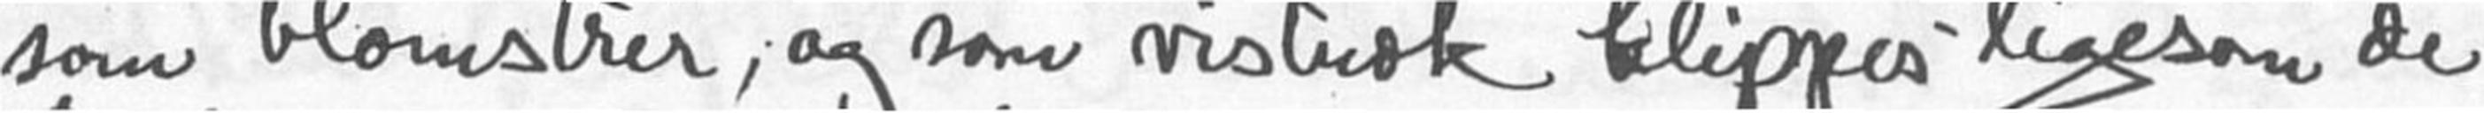som blomstrer, og som vistnok klippes ligesom de
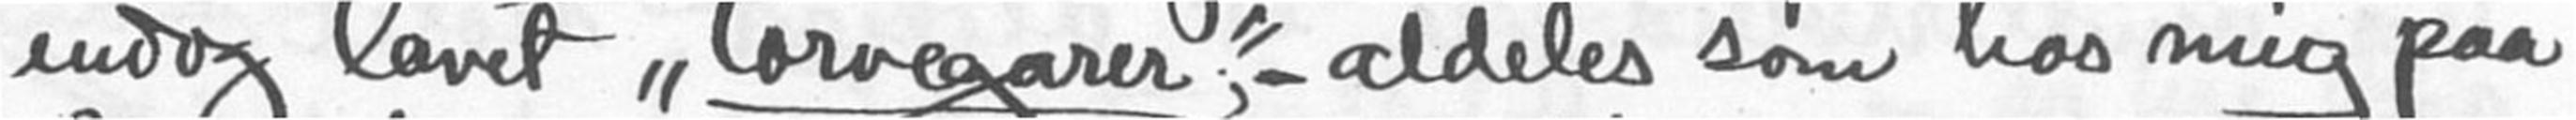endog lavet "torvegarer"; - aldeles som hos mig paa
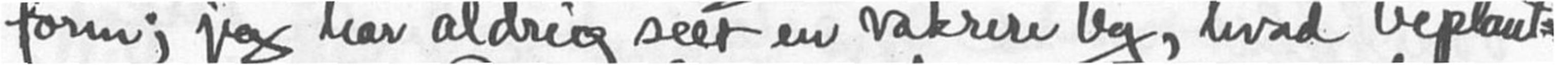form; jeg har aldrig seet en vakrere by, hvad beplant-
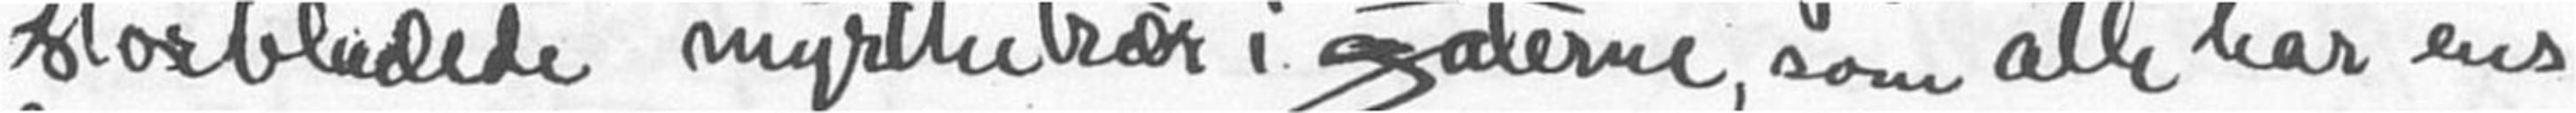storbladede myrthetrær i gaterne, som alle har ens
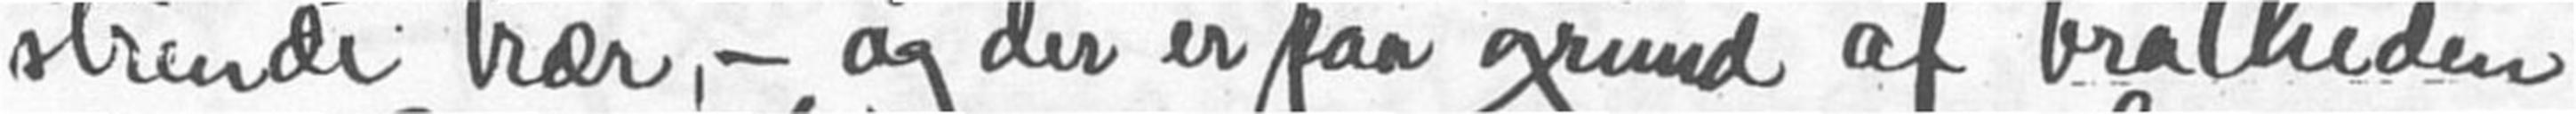strende trær, - og der er paa grund af bratheden
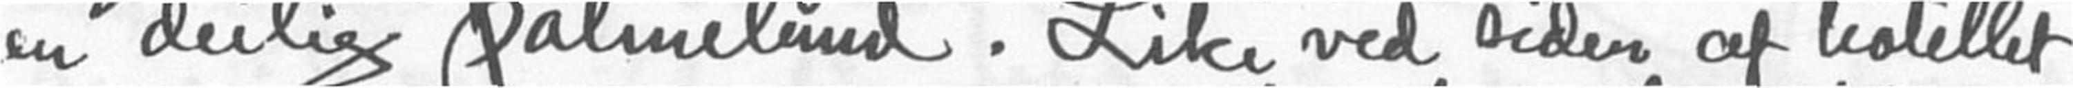en deilig palmelund. Like ved siden af hotellet
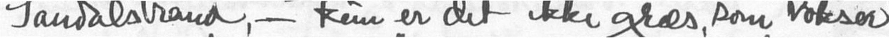Sandalstrand, - kun er det ikke græs, som vokser
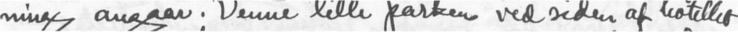ning angaar. Denne lille parken ved siden af hotellet
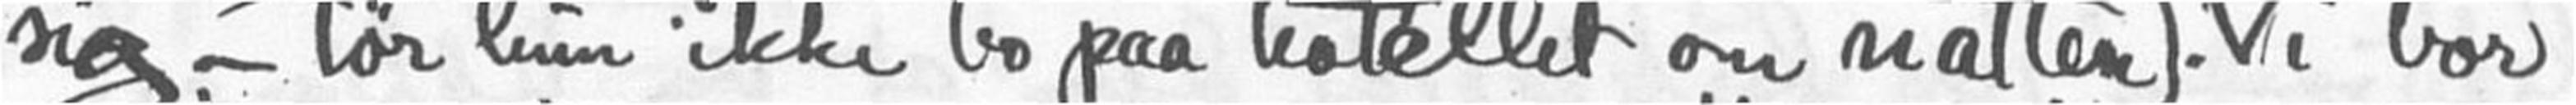sig,- tør hun ikke bo paa hotellet om natten). Vi bor
